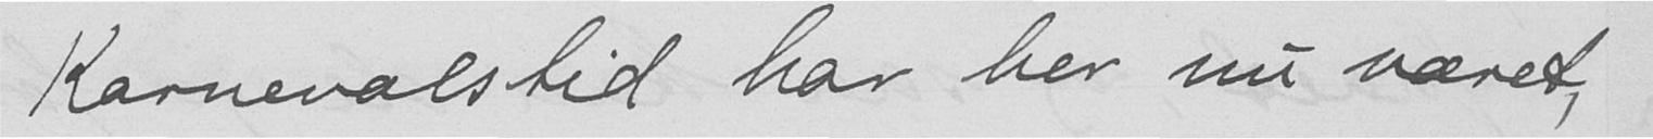Karnevalstid har her nu været
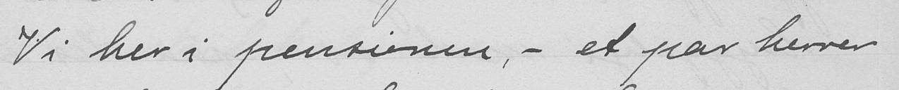Vi her i pensionen,- et par herrer
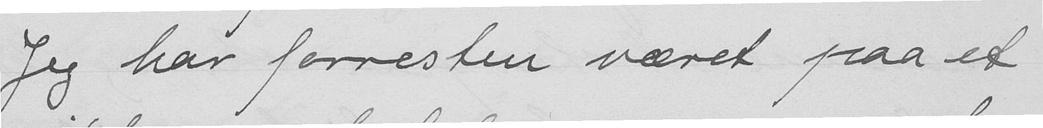Jeg har forresten været paa et
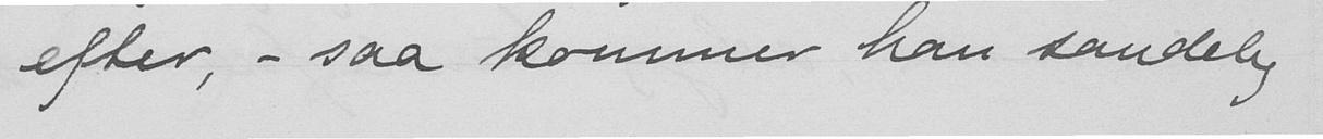efter, - saa kommer han sandelig
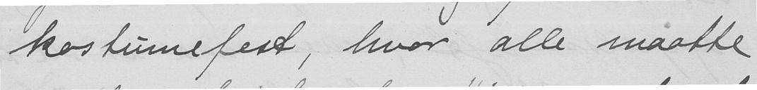kostumefest, hvor alle maatte
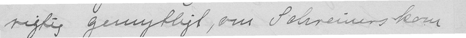rigtig gemytligt, om Schreiners kom
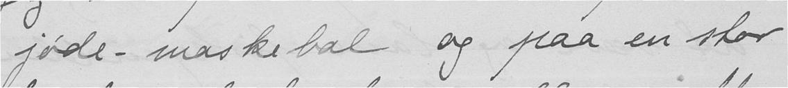jøde - maskebal og paa en stor
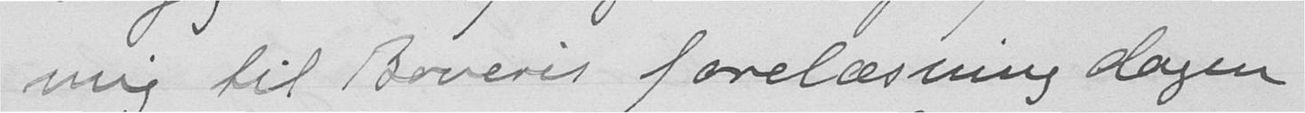mig til Boveris forelæsning dagen
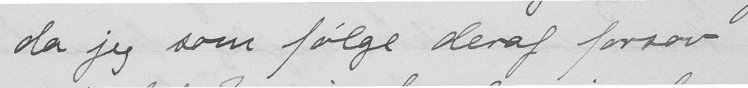da jeg som følge deraf forsov
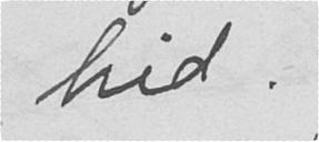hid.
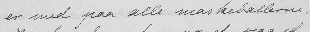er med paa alle maskeballerne.
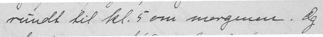rundt til kl. 5 om morgenen. og
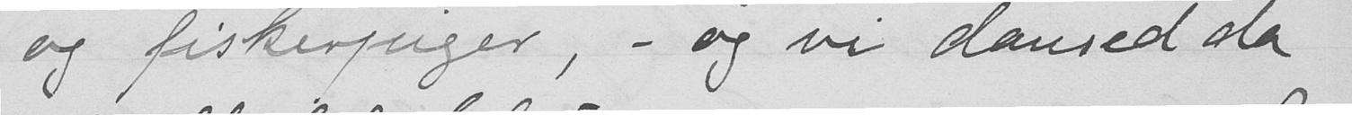og fiskerpiger, - og vi dansed da
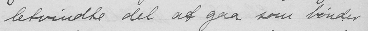letvindte del at gaa som bønder
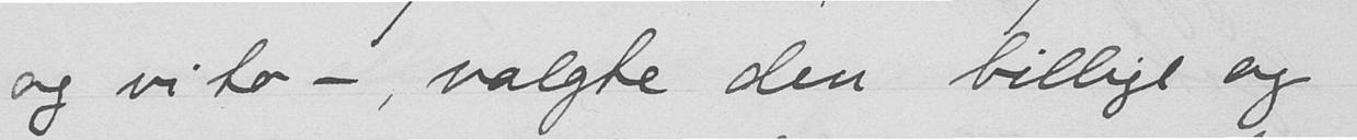og vi to -, valgte den billige og
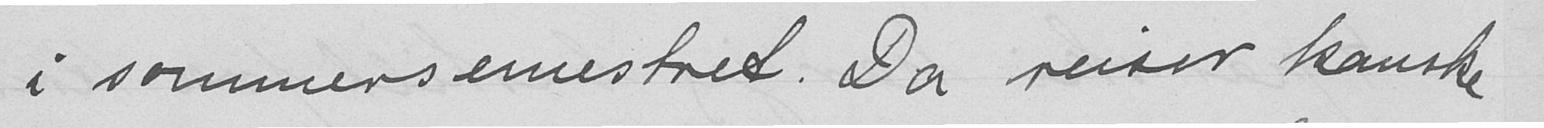i sommersemestret. Da reiser kanske
